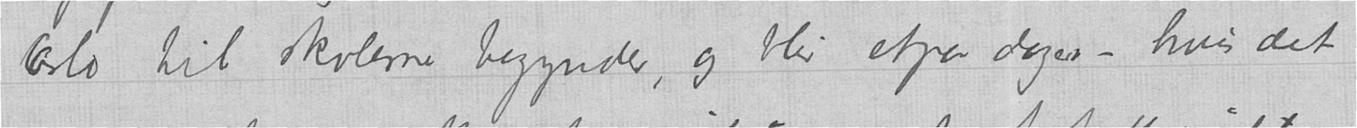Oslo til skolerne begynder, og blir etpar dager – hvis det
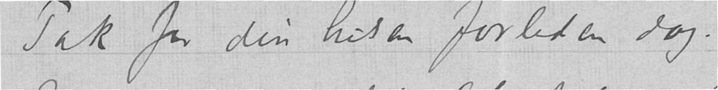Tak for din hilsen forleden dag.
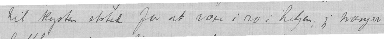til kysten etsted for at være i ro i helgen; jeg trænger
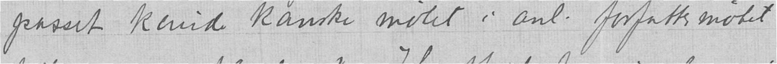passet kunde kanske møtet i anl. forfattermøtet
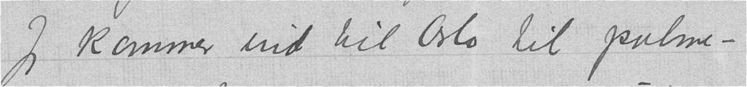Jeg kommer ind til Oslo til palme-
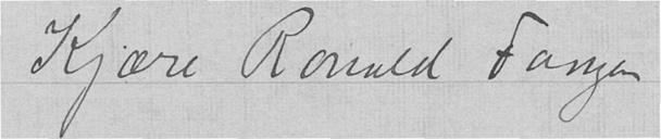Kjære Ronald Fangen
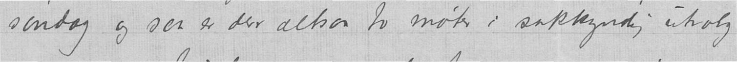søndag og saa er der altsaa to møter i sakkyndig utvalg
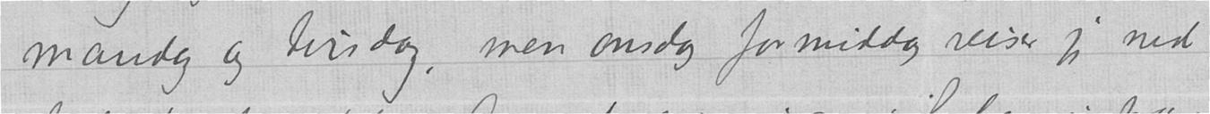mandag og tirsdag, men onsdag formiddag reiser jeg ned
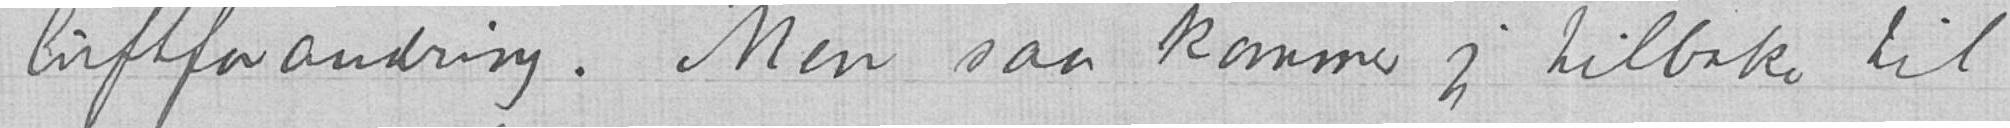luftforandring. Men saa kommer jeg tilbake til
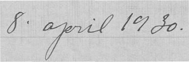8. april 1930.
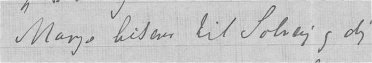Mange hilsener til Solveig og dig
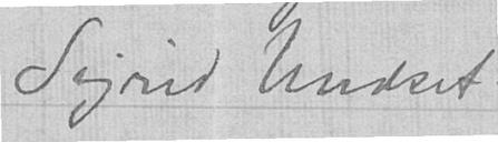Sigrid Undset.
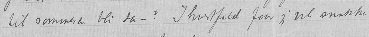til sommeren bli da –? I hvertfald faar jeg vel snakke
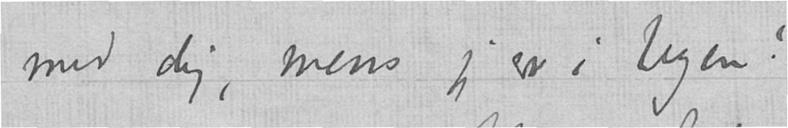med dig, mens jeg er i byen?
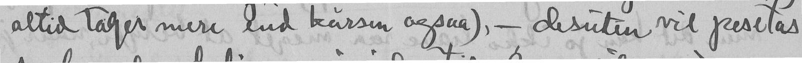altid tager mere end kursen ogsaa), - desuten vil pesetas
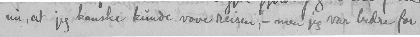nu, at jeg kanske kunde vove reisen, - men jeg var bedre for
